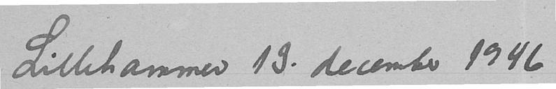Lillehammer 13. december 1946
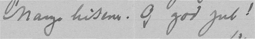Mange hilsener. Og god jul!
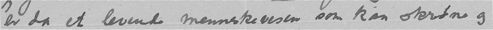er da et levende menneskevesen som kan skrøne og
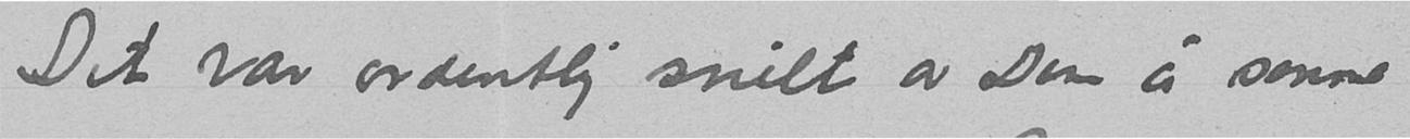Det var ordentlig snilt av Dem å senne
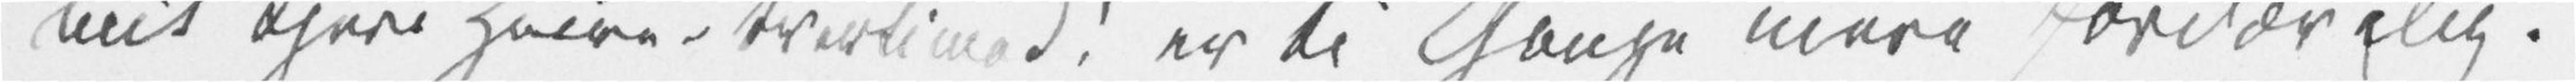mit kjere «Høire» – tvertimod! er ti Gange mere fordæ[r]velig.
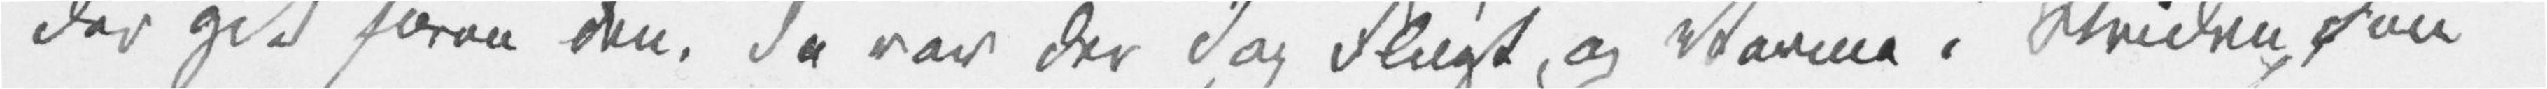der gik foran den. Da var der dog Flugt og Varme i Striden, den
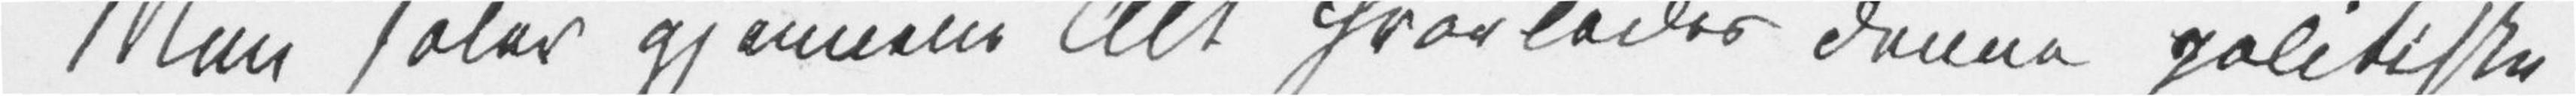Man føler gjennem Alt hvorledes denne politiske
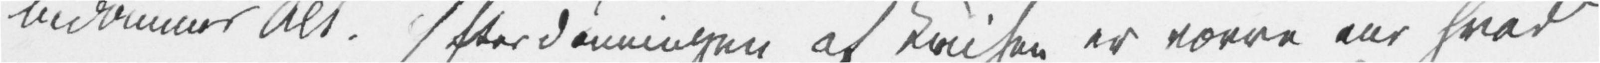bedømmes Alt. Efterdønningen af Krisen er værre end hvad
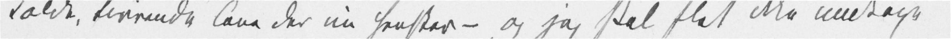kolde, tirrende Tone der nu hersker – og jeg skal slet ikke undtage
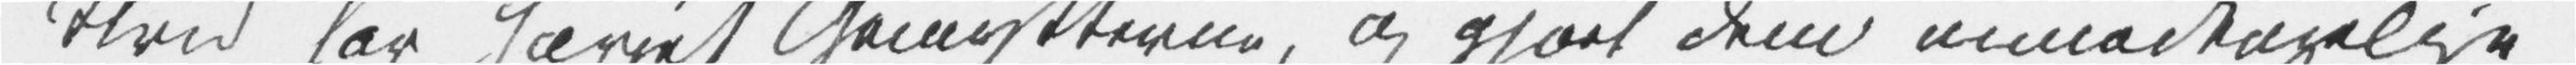Strid har hærjet Gemytterne, og gjort dem uimodtagelige
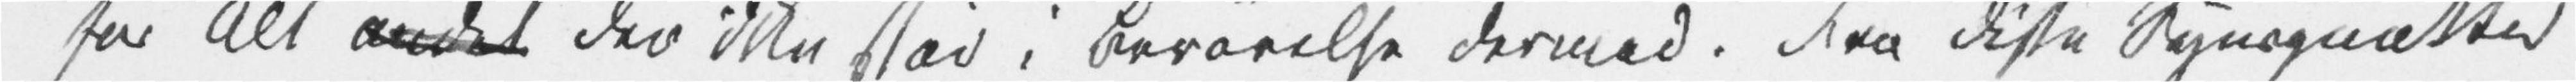for Alt andet der ikke står i Berørelse dermed. Fra disse Synspunkter
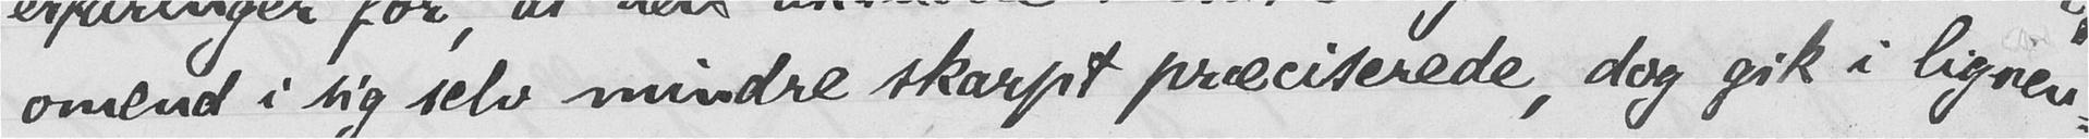omend i sig selv mindre skarpt præciserede, dog gik i lignen-
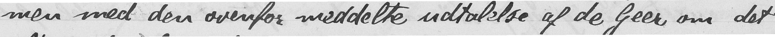men med den ovenfor meddelte udtalelse af de Geer om det
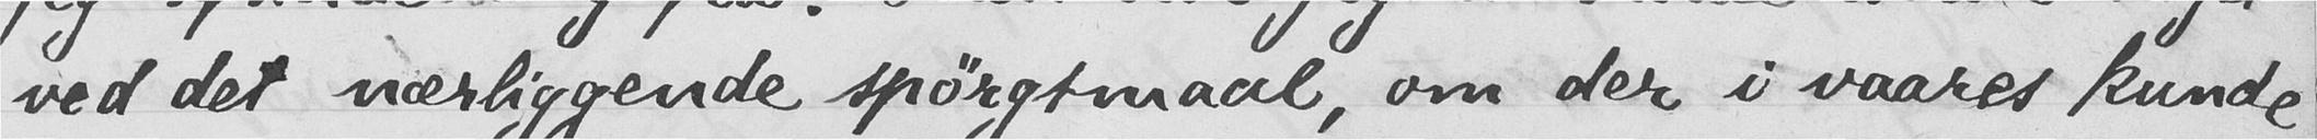ved det nærliggende spørgsmaal, om der i vaares kunde
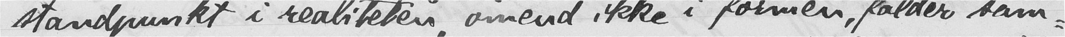standpunkt i realiteten, omend ikke i formen, falder sam-
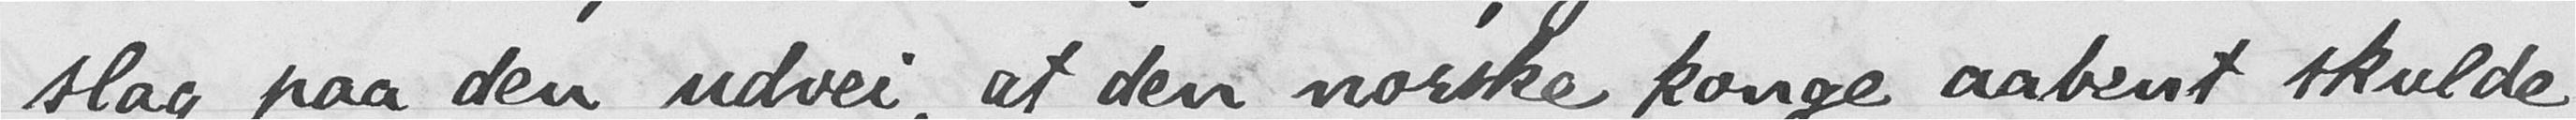slag paa den udvei, at den norske konge aabent skulde
